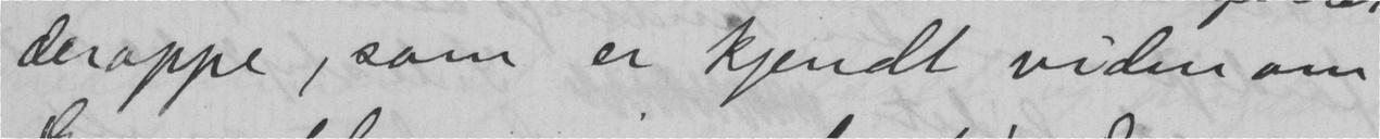deroppe, som er kjendt viden om
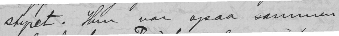styret. Hun var ogsaa sammen
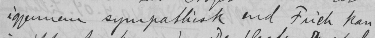igjennem sympatisk end Frich kan
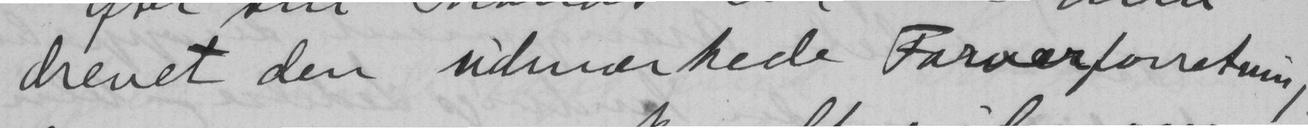drevet den udmærkede Farverforretning
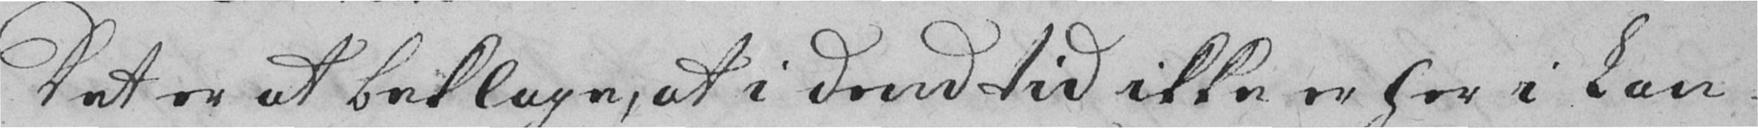Det er at beklage, at i dend tid ikke er her i Lan-
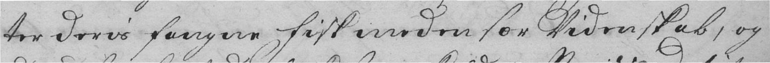ter deris fangne Fisk med en sær Videnskab, og
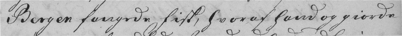Bergen fangede Fisk, hvoraf hand og giorde
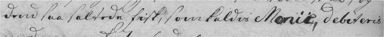dend saa saltede Fisk, som kaldis Monie, debiteris
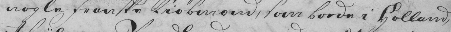nogle Franske Kiøbmænd, som boede i Holland,
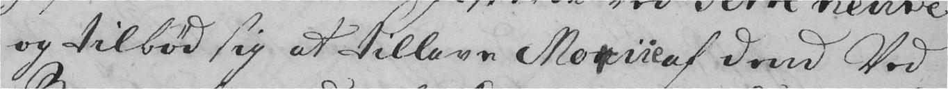og tilbød sig at tillave Monie af dend Ved
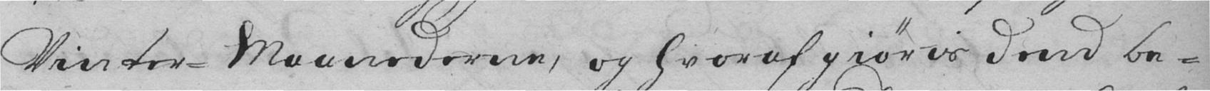Vinter-Maanederne, og hvoraf giøris dend be-
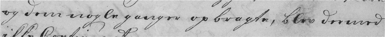og dem nogle ganger opbragte, blev dermed
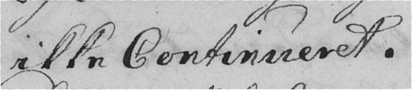ikke Continneret.
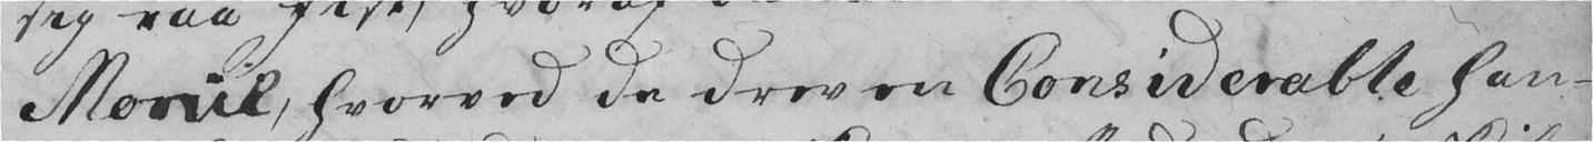Monie, hvorved de drev en Considerable han-
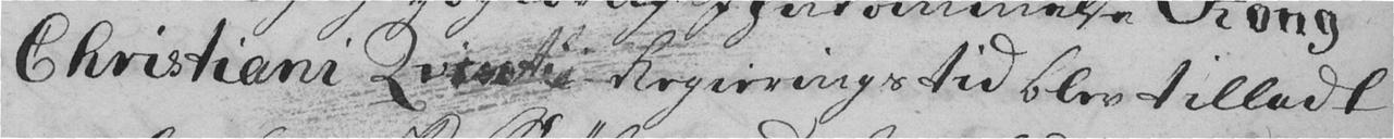Christiani Qvinti Regieringstid blev tilladt
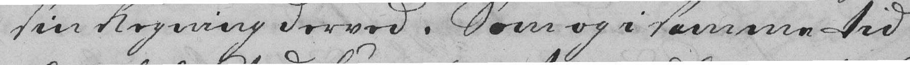sin Regning derved. Som og i samme tid
